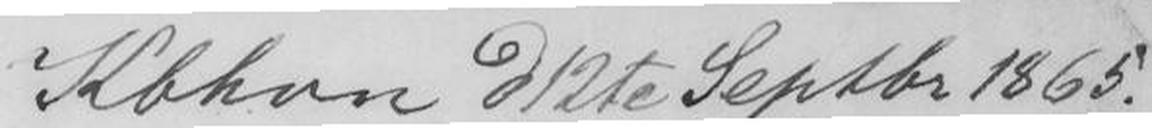Kbhvn d 12te Septbr 1865.
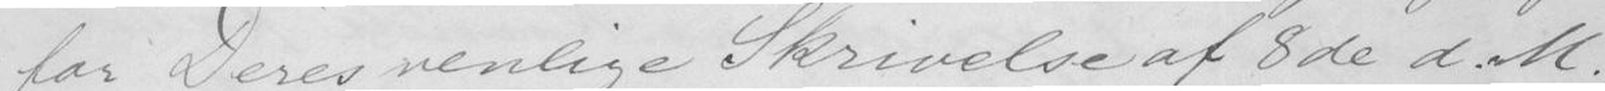for Deres venlige Skrivelse af 8 de d. M.,
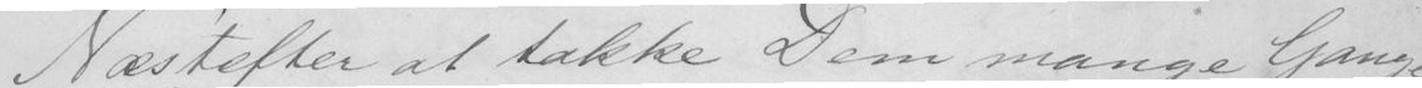Næstefter at takke Dem mange Gange
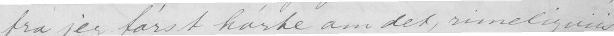fra jeg først hørete om det, rimeligviis
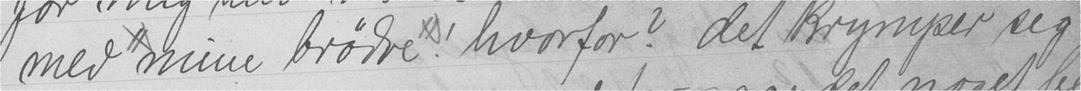med mine brødre"! hvorfor? det krymper sig
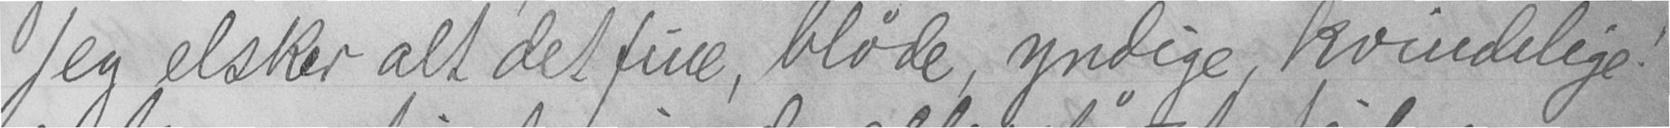Jeg elsker alt det fine, bløde yndige, kvindelige!
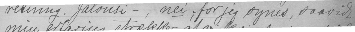retning. Jalousi - , nei; for jeg synes, saavidt
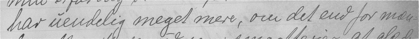har uendelig meget mere, om det end for mæn-
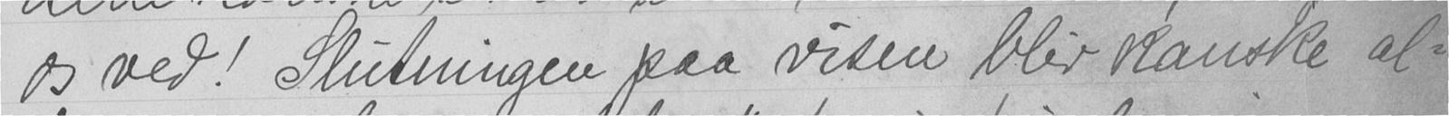os ved! Slutningen paa visen blir kanske al-
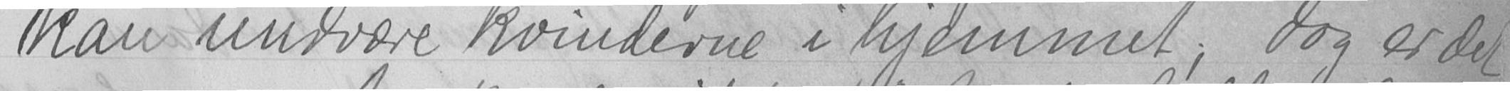kan undvære kvinderne i hjemmet; dog er det
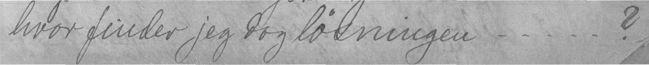hvor finder jeg dog løsningen - - - - - ?
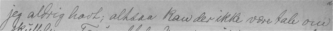jeg aldrig havt; altsaa kan der ikke være tale om
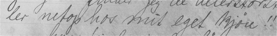ler netop hos mit eget kjøn!!
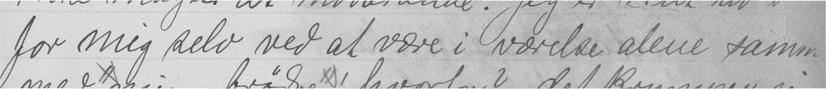for mig selv ved at være i værelse alene sammen
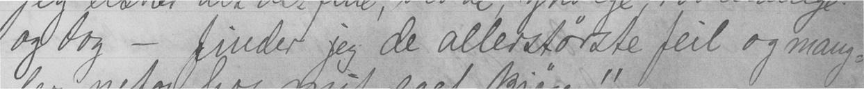og dog - finder jeg de allerstørste feil og mang-
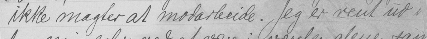ikke magter at modarbeide. Jeg er rent ud l
